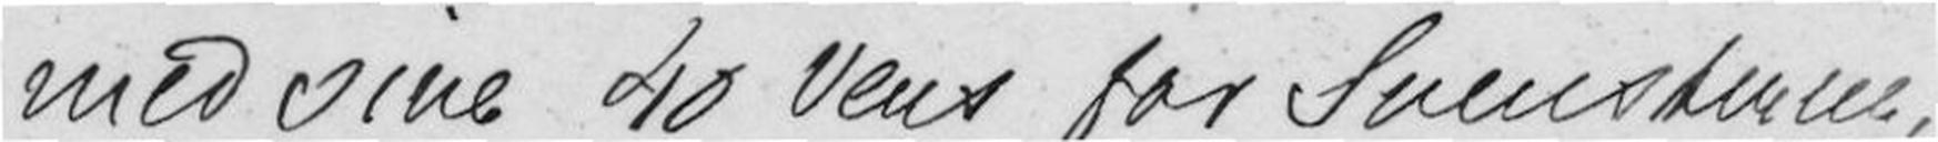med sine 40 vers for Svenskerne,
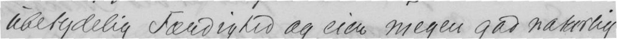ubetydelig Færdighed og eier megen god makerlig
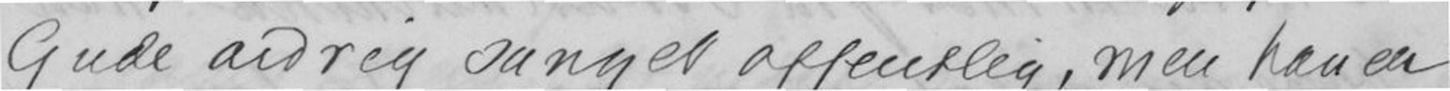Gude aldrig sanget offentlig, men han er
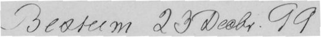Bestum 23 Desbr. 99
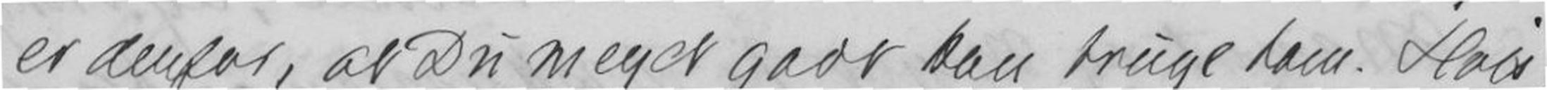er derfor, at Du meget godt kan truge ham. Holt
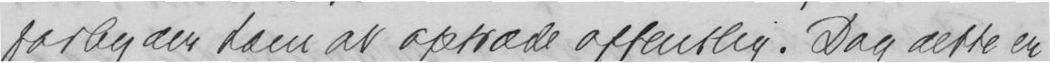forbyder ham at optræde oppffentlig. Dag dette er
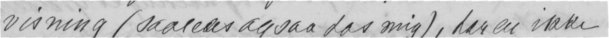visning (saaledes ogsaa hos mig), har en ikke
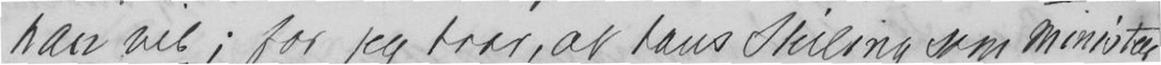han vil; for jeg tror, at hans Skildring som ministær
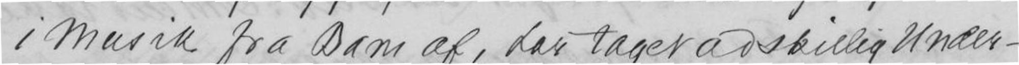i Musik fra Barn af, han tager adskillig Under-
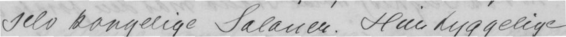selv kongelige Salaner. Har hyggelige
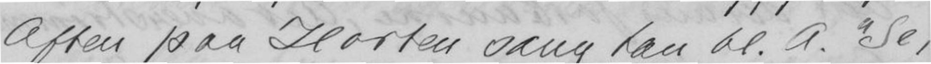Aften paa Horten sang han bl.a. "Se,
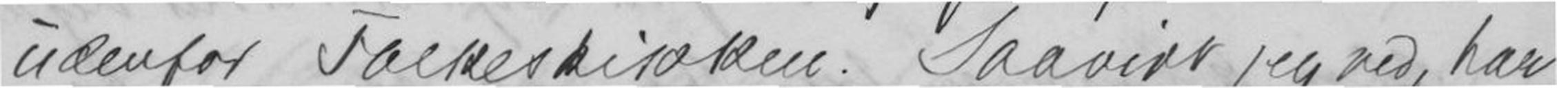udenfor Folkeskikken. Saavidt jeg ved, har
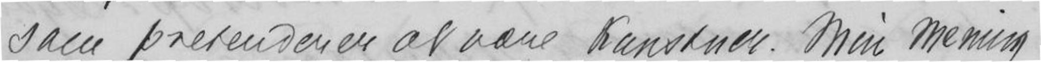som presenderer at være Kunstnar. Min Mening
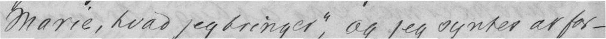Marie, hvad jeg Bringer", og jeg syntes at for-
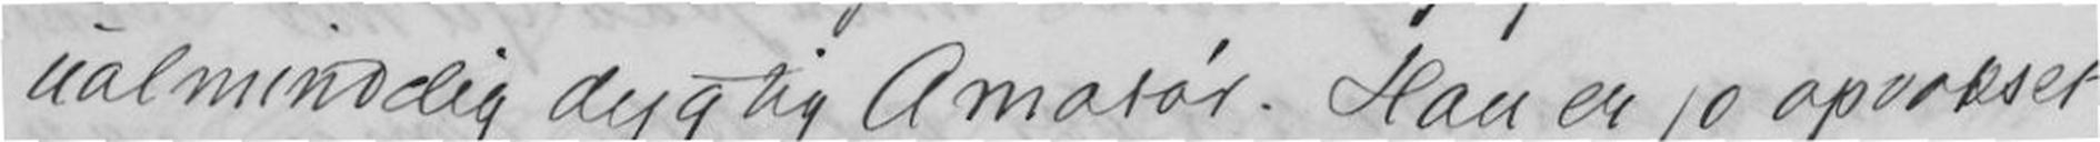ualminedig dygtig Amatør. han er jo opvokset
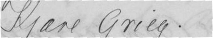Kjære Grieg
